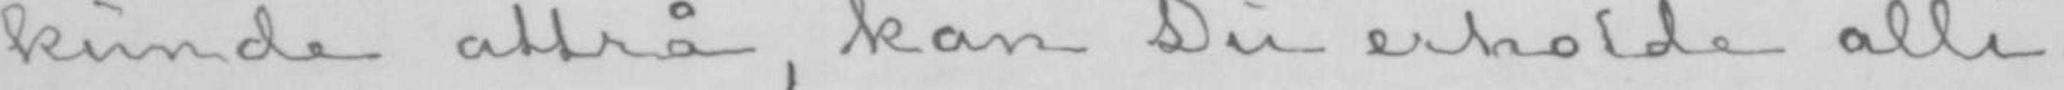kunde attrå, kan Du erholde alli-
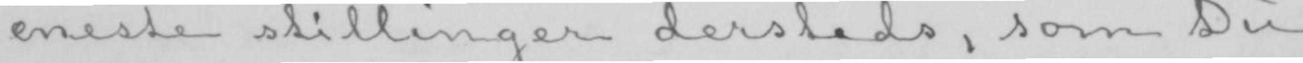eneste stillinger dersteds, som Du
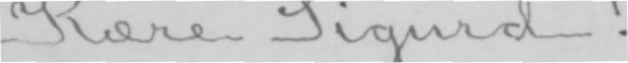Kære Sigurd!
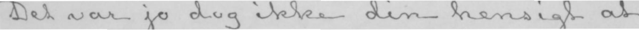Det var jo dog ikke din hensigt at
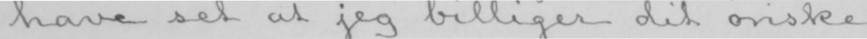have set at jeg billiger dit ønske
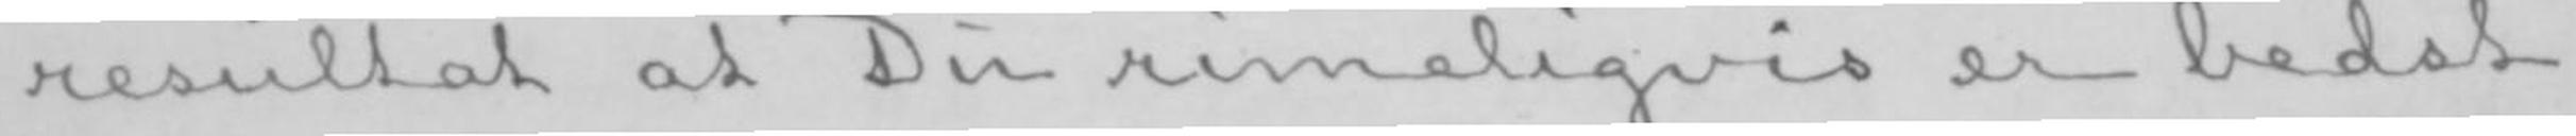resultat at Du rimeligvis er bedst
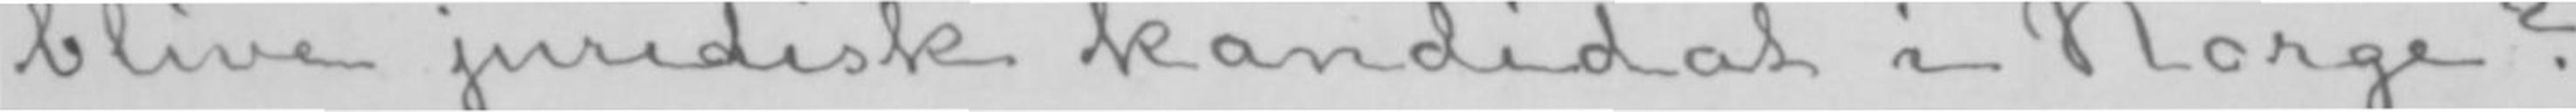blive juridisk kandidat i Norge?
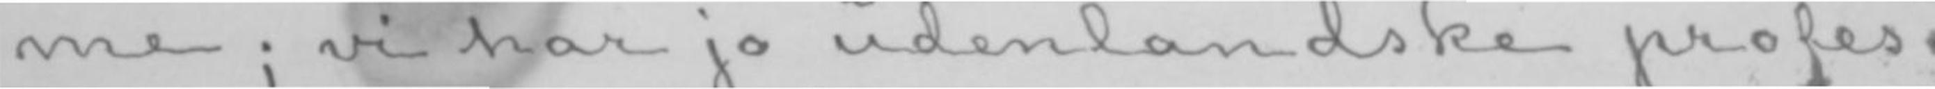me; vi har jo udenlandske profes-
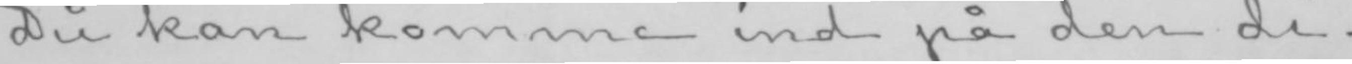Du kan komme ind på den di-
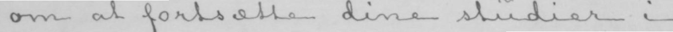om at fortsætte dine studier i
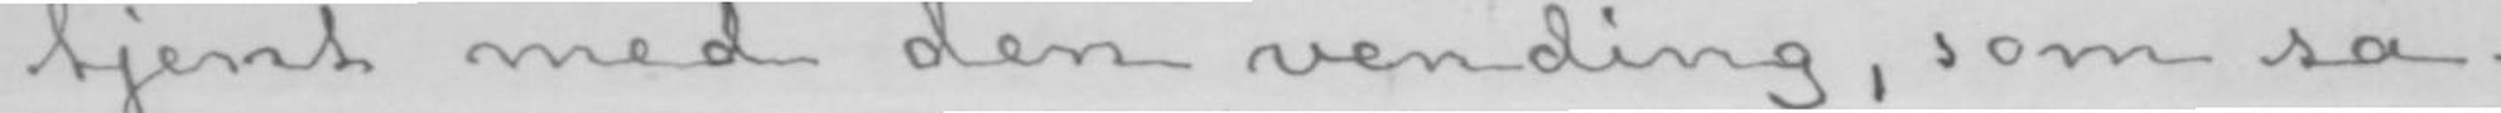tjent med den vending, som sa-
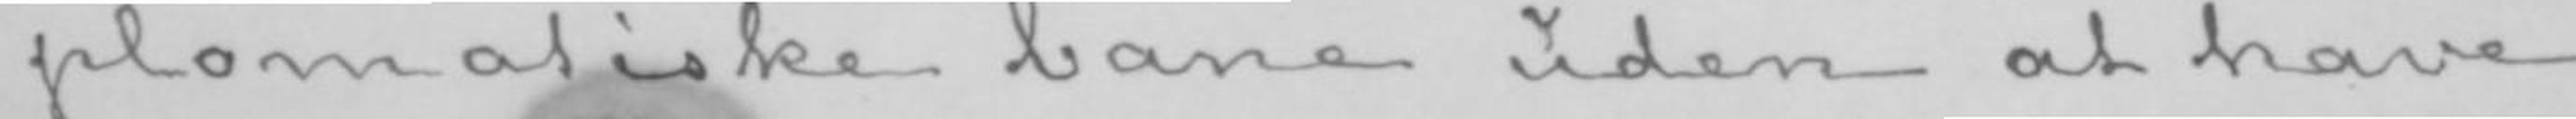plomatiske bane uden at have
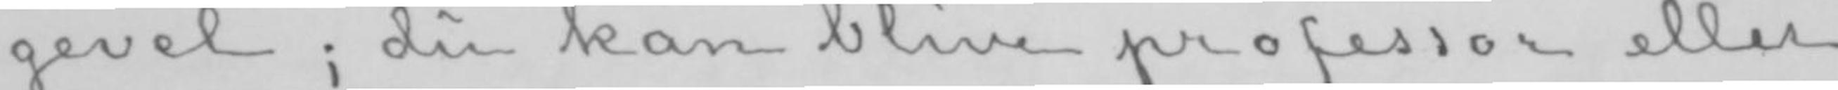gevel; du kan blive professor eller
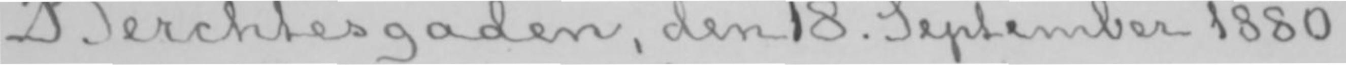Berchtesgaden, den 18. September 1880.
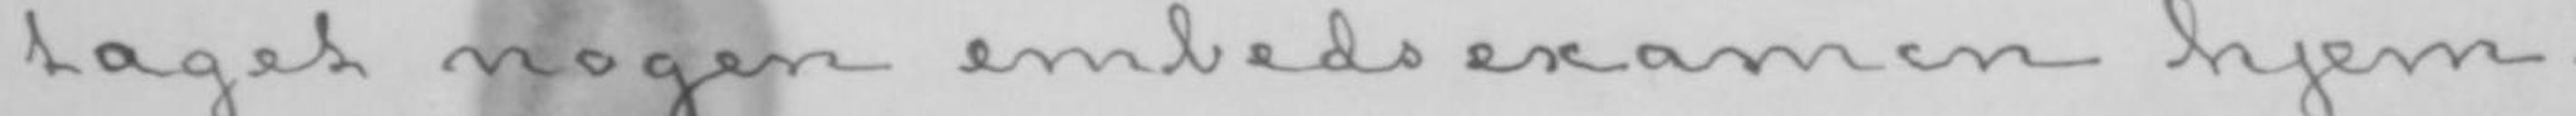taget nogen embedsexamen hjem-
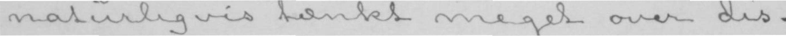naturligvis tænkt meget over dis-
